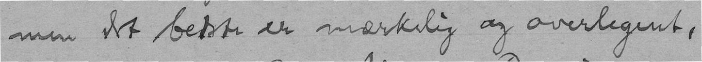men det bedste er mærkelig og overlegent,
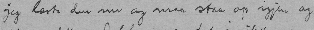jeg læste den nu og maa staa op igjen og
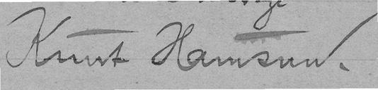Knut Hamsun.
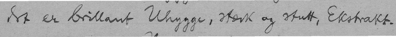det er brillant Uhygge, stærk og stutt, Ekstrakt.
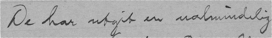De har utgit en ualmindelig
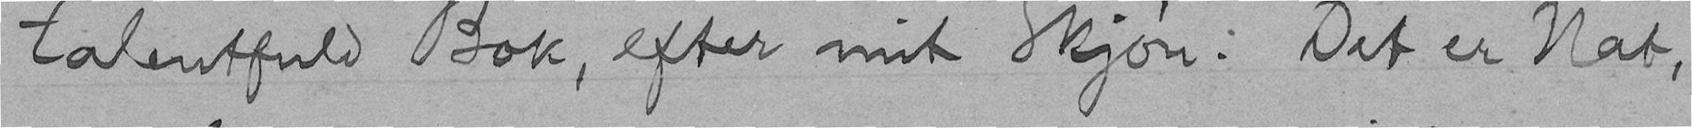talentfuld Bok, efter mit Skjøn: Det er Nat,
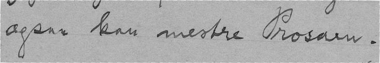ogsaa kan mestre Prosaen.
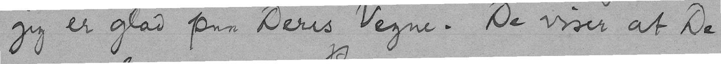jeg er glad paa Deres Vegne. De viser at De
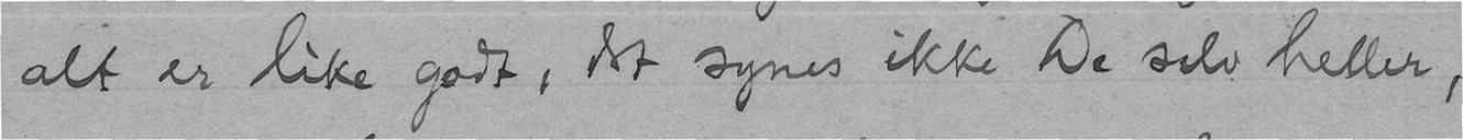alt er like godt, det synes ikke De selv heller,
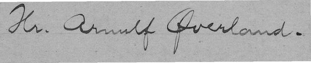Hr. Arnulf Øverland.
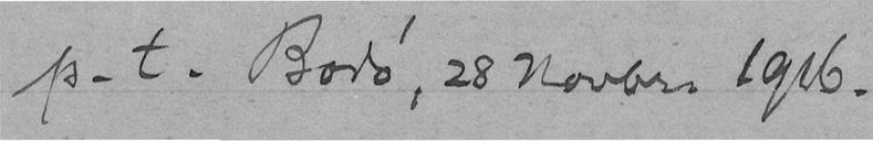p.t. Bodø, 28 Novbr. 1916.
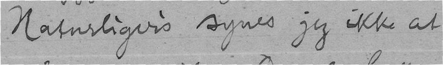Naturligvis synes jeg ikke at
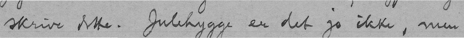skrive dette. Julehygge er det jo ikke, men
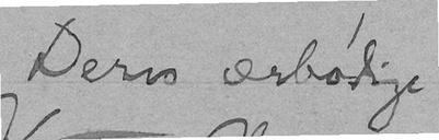Deres ærbødige
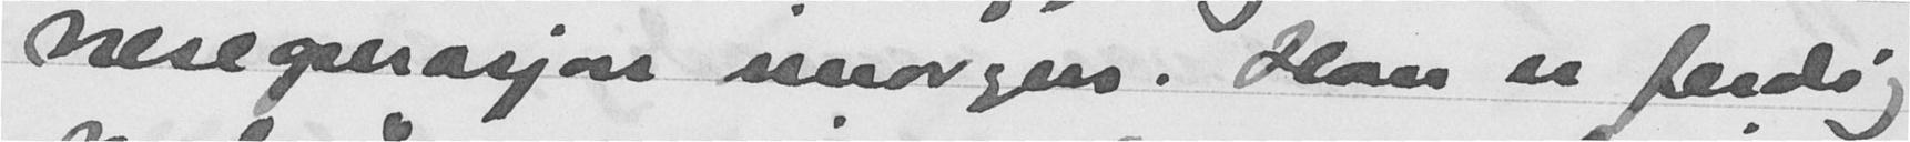neseoperasjon imorgen. Han er ferdig
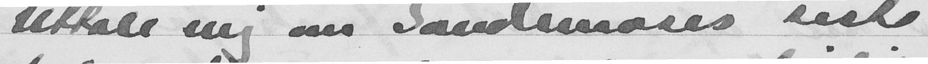Uttale mig om Sandemoses siste
